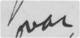var
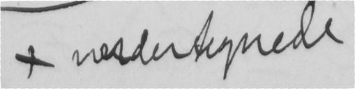x undertegnede
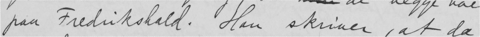paa Fredrikshald. Han skriver, at da
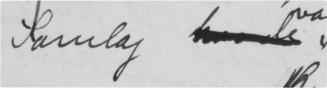Samlag
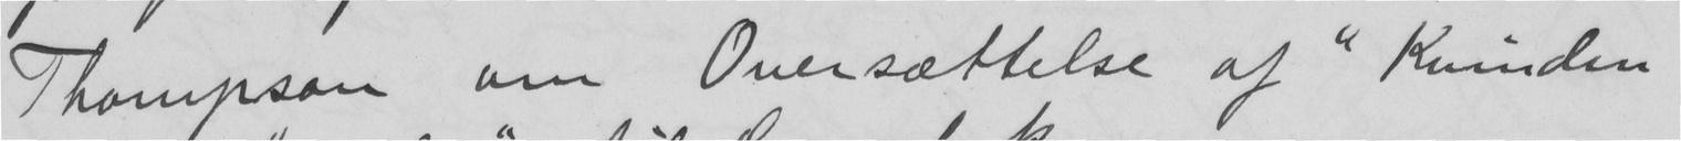Thompson om Oversættelse af "Kvinden
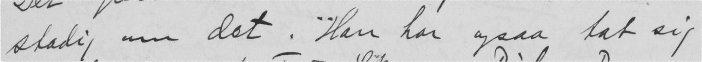stadig om det. Han har ogsaa tat sig
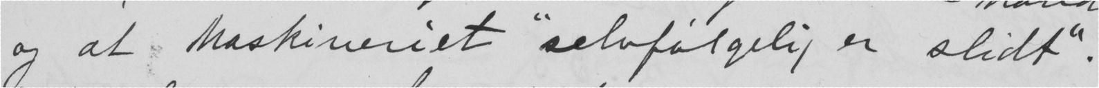og at Maskineriet "selvfølgelig er slidt".
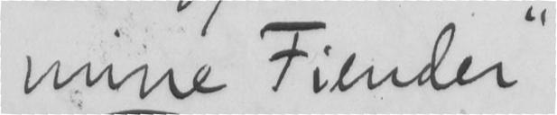mine Fiender"
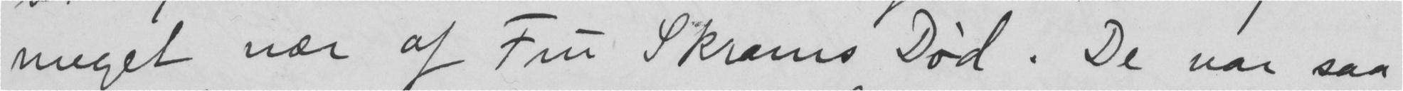meget nær af Fru Skrams Død. De var saa
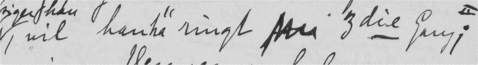vil han ha "ringt  3die gang";
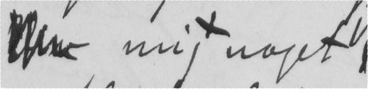mig noget
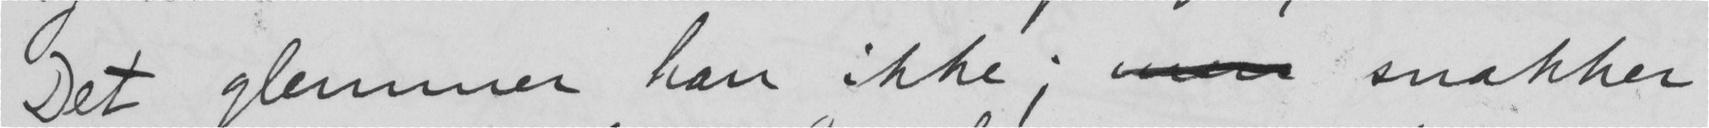Det glemmer han ikke;  snakker
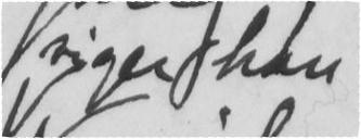siger han
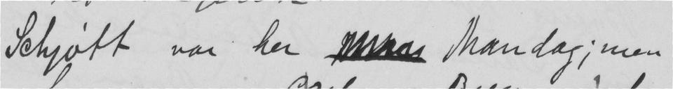Schjøtt var her  Mandag; men
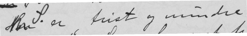S. er trist og mindre
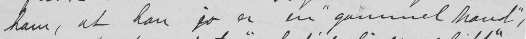ham, at han jo er en "gammel Mand",
